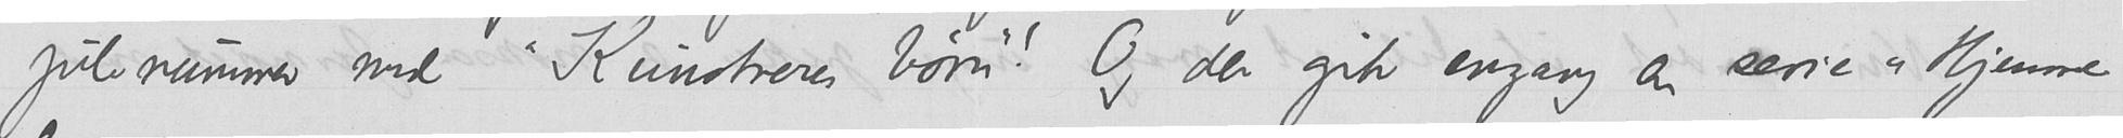julenummer med "Kunstneres børn"! Og der gik engang en serie "Hjemme
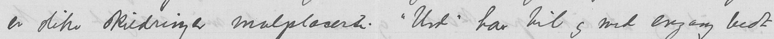er slike skildringer malplaserte. "Urd" har til og med engang bedt
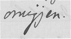omigjen.
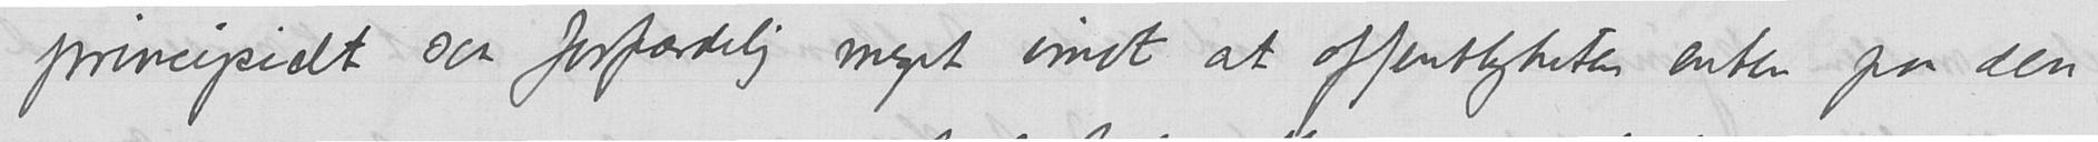principielt saa forfærdelig meget imot at offentligheten enten paa den
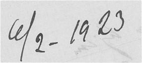6/2-1923
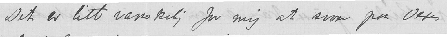Det er litt vanskelig for mig at svare paa Deres
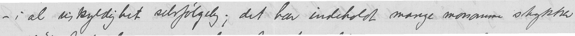– i al uskyldighet selvfølgelig; det har indeholdt mange morsomme stykker
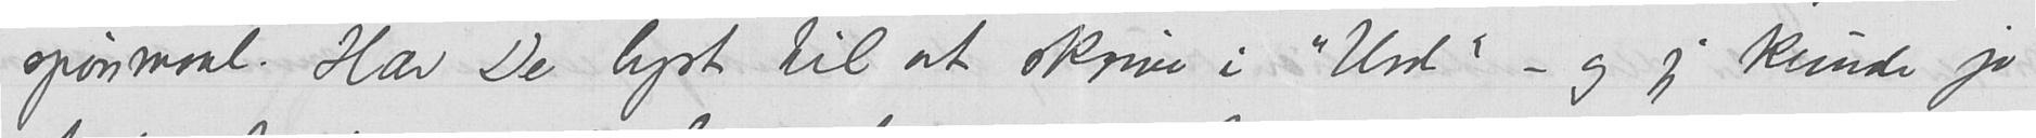spørsmaal. Har De lyst til at skrive i «Urd» – og jeg kunde jo
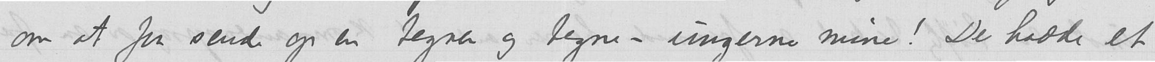om at faa sende op en tegner og tegne – ungerne mine! De hadde et
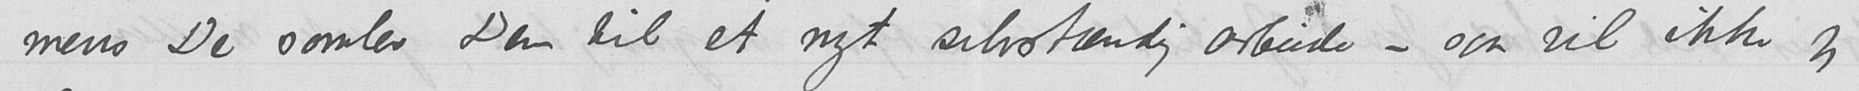mens De somler Dem til et nyt selvstændig arbeide – saa vil ikke jeg
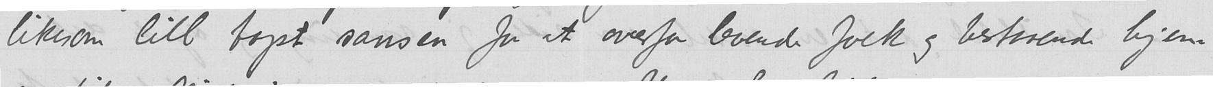likesom litt tapt sansen for at overfor levende folk og bestaaende hjem
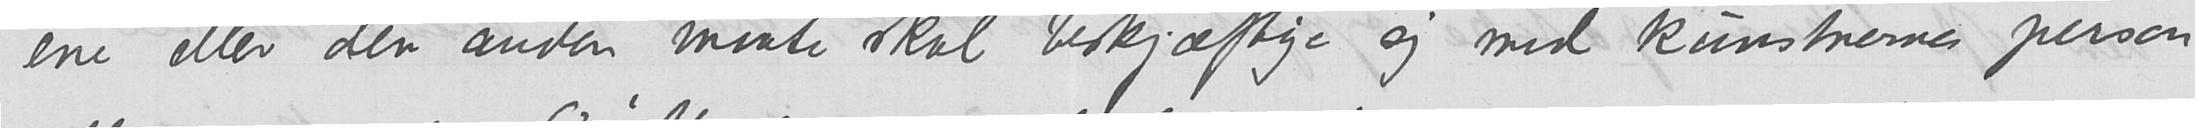ene eller den anden maate skal beskjæftige sig med kunstnernes person
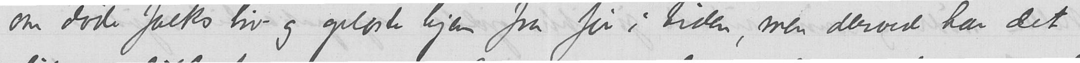om døde folks liv og opløste hjem fra før i tiden, men derved har det
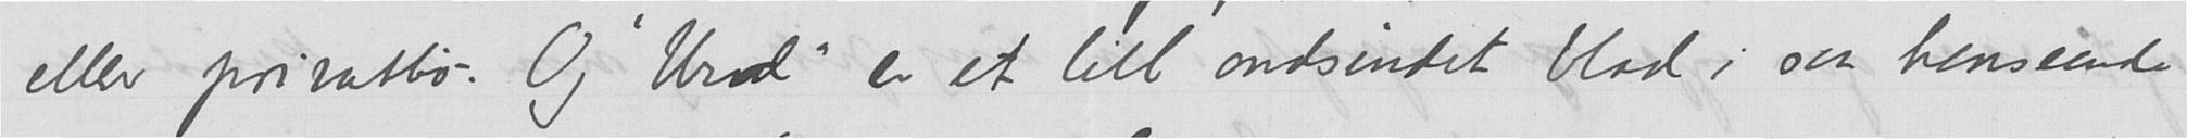eller privattiv. Og "Urd" er et litt ondsindet blad i saa henseende
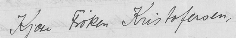Kjære Frøken Kristofersen,
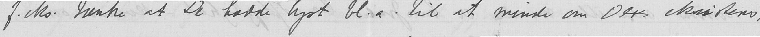f.eks. tænke at De hadde lyst bl.a. til at minde om Deres eksistens,
